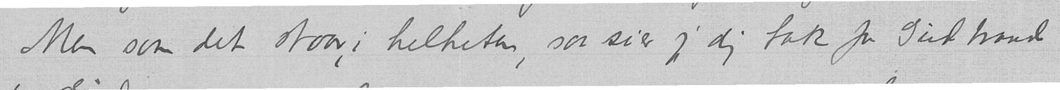Men som det staar, i helheten, saa sier jeg dig tak for Gudbrand
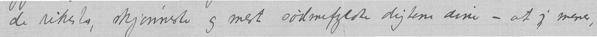de rikeste, skjønneste og mest sødmefyldte digtene dine – at jeg mener,
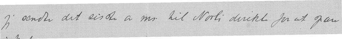jeg sendte det sisste av ms. til Norli direkte for at spare
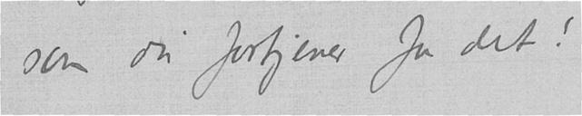som du fortjener for det!
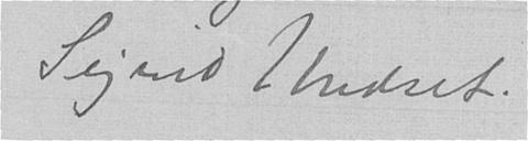Sigrd Undset.
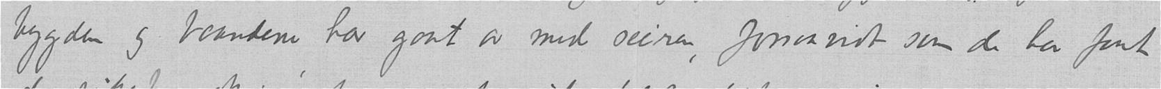bygden og baandene har gaat av med seiren, forsaavidt som de har faat
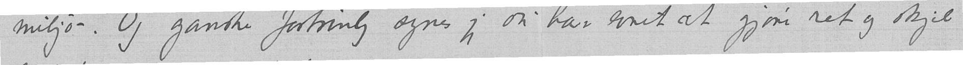miljø –. Og ganske fortrinlig synes jeg du har evnet at gjøre ret og skjel
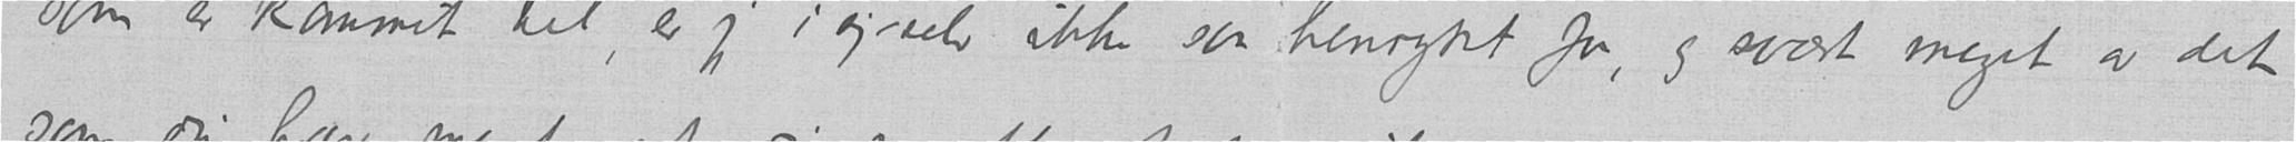som er kommet til, er jeg i sig selv ikke saa henrykt for, og svært meget av det
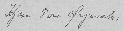Kjære Tore Ørjasæter,
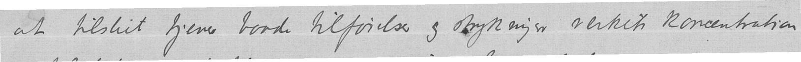at tilslut tjener baade tilføielser og strykninger verkets koncentration
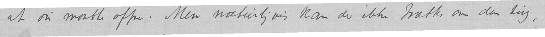at du maatte offre. Men naturligvis kan der ikke trættes om den ting,
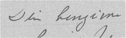Din hengivne
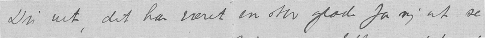Du vet, det har været en stor glæde for mig at se
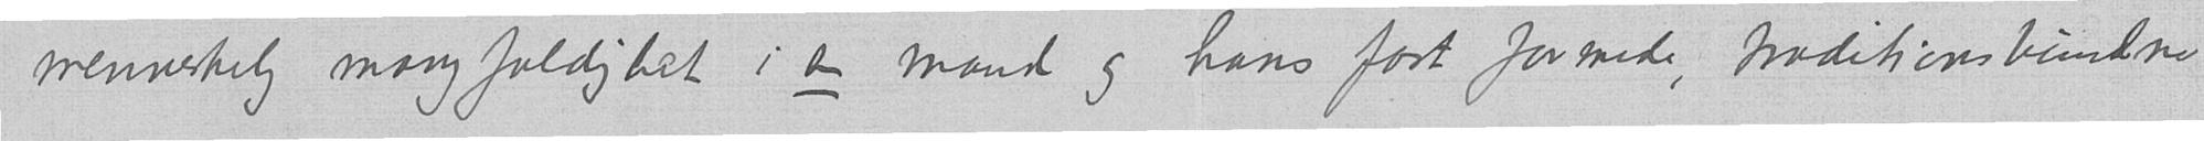menneskelig mangfoldighet i en mand og hans fast formede, traditionsbundne
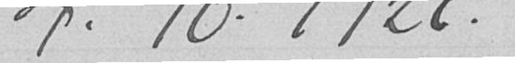4. 10. 1926.
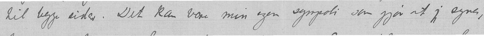til begge sider. Det kan være min egen sympati som gjør at jeg synes,
